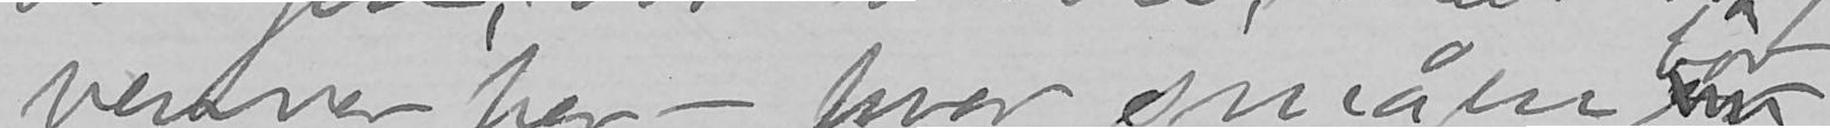venner her - hvor småen er
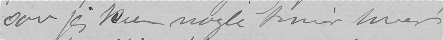sov jeg kun nogle timer hver
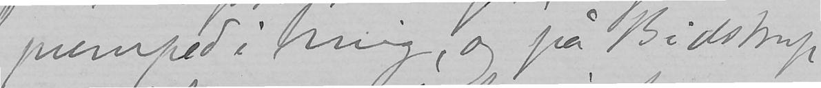pumped i mig, og på Bidstrup,
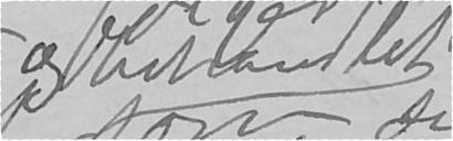og behandlet
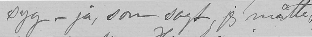syg, - ja, som sagt, jeg måtte
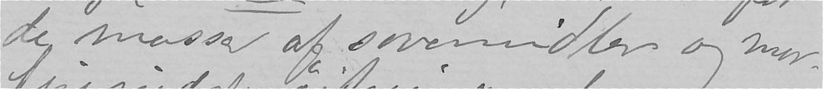de masser af sovemidler og mor-
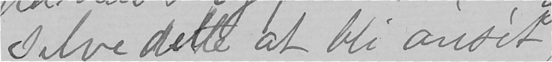selve dette at bli ansét
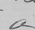og
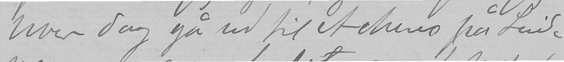hver dag gå ud til Achens på Linde-
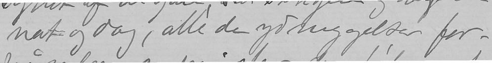nat og dag, alle de ydmygelser for-
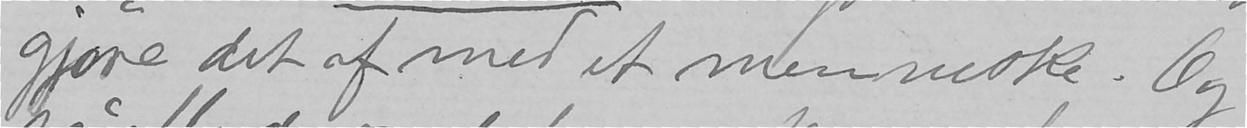gjøre det af med et menneske. Og
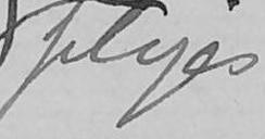plejes
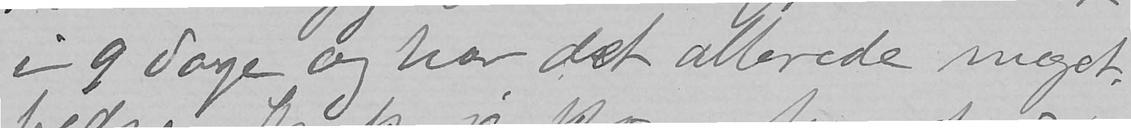i 9 dage, og har det allerede meget
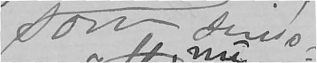som sind-
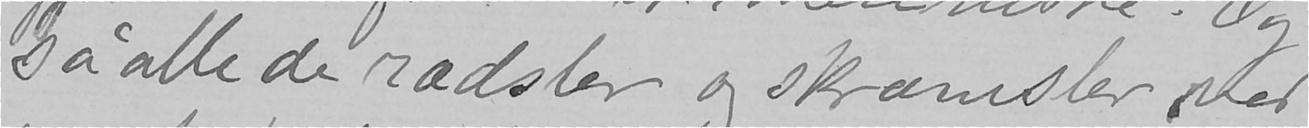så alle de rædsler og skræmsler ved
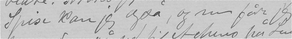Spise kan jeg også, og nu får jeg
